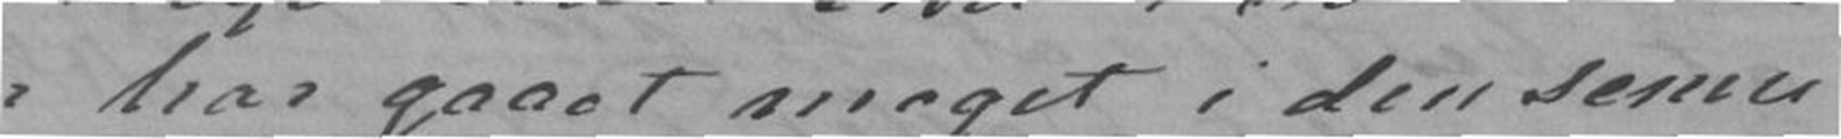har gaaet meget i den senere
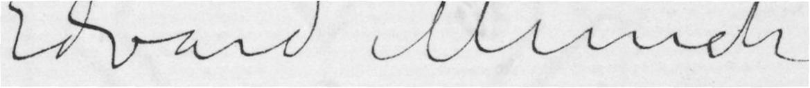Edvard Munch
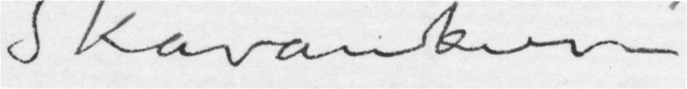Skavanker –
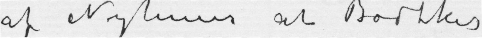af Nyhuus at Bødtker
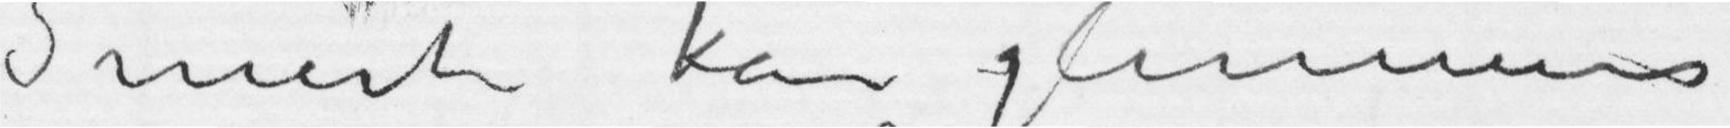Smerte kan glemmes
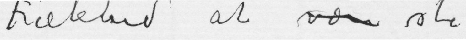Frækhed at være stå
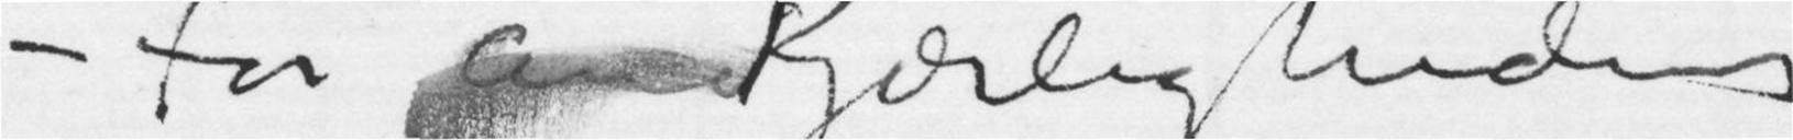– For aKjærlighedens
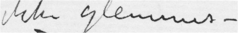ikke glemmes –
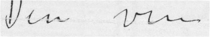Din ven
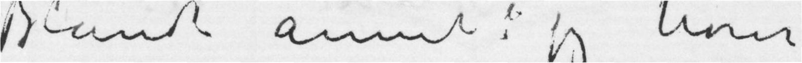Blandt annet: jeg hører
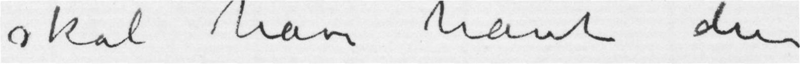skal have havt den
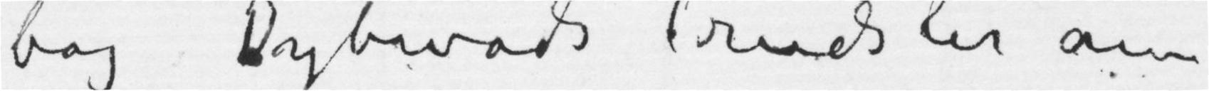bag Dybwads Trudsler om
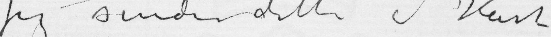Jeg sender dette i Hast
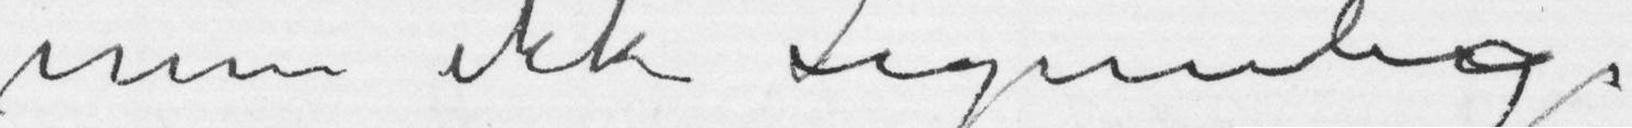men ikke Legemlige
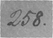258.
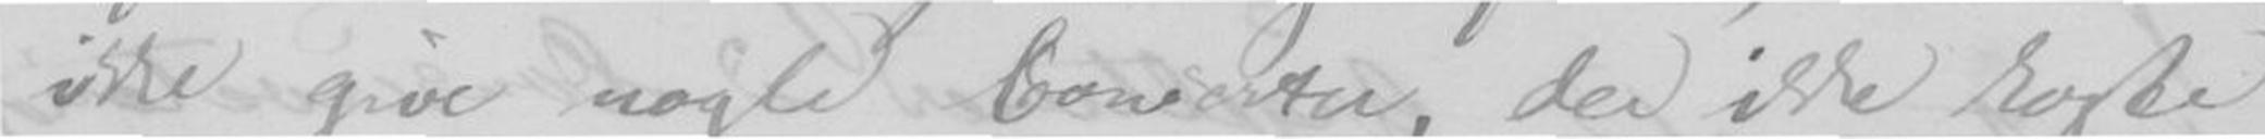ikke give nogle Conserter, der ikke koste
